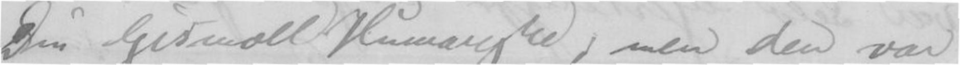Din Gismoll Humoreske, men den var
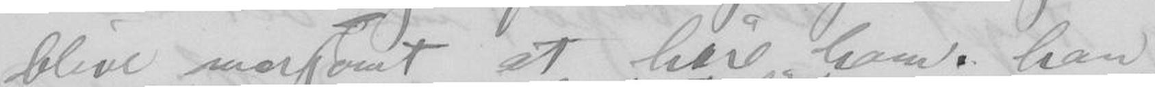blive morsomt at høre ham; han
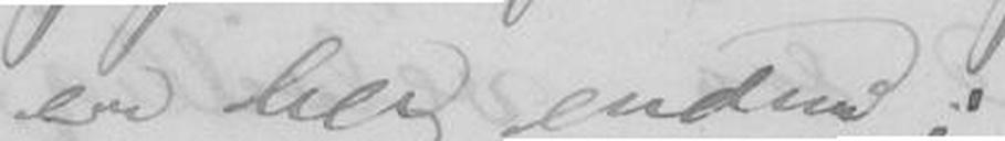er her endnu;
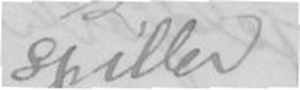spiller
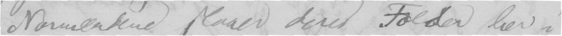Normændene slaar deres Folder her i
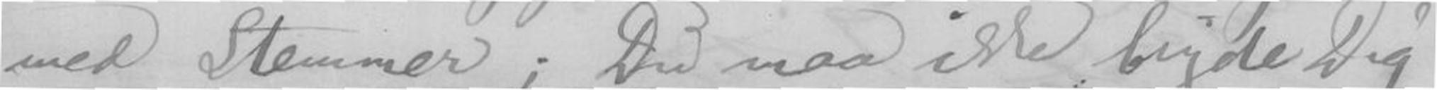med Stemmer; Du maa ikke bryde Dig
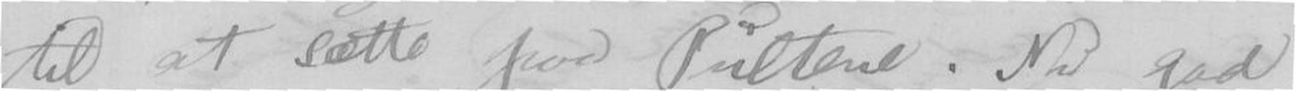til at sætte paa Pultene. Nu gad
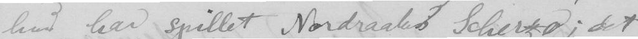hun har spillet Nordraaks Scherzo; det
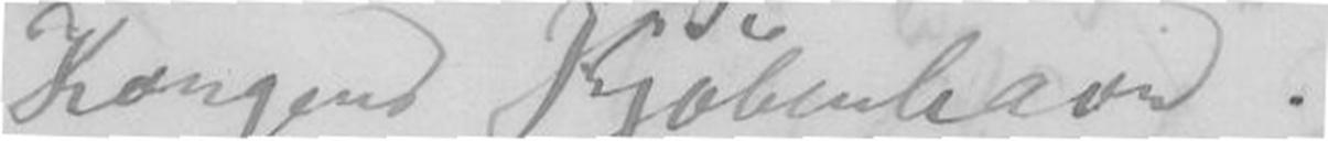Kongens Kjøbenhavn.
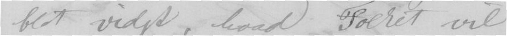blot vidst, hvad Folket vil
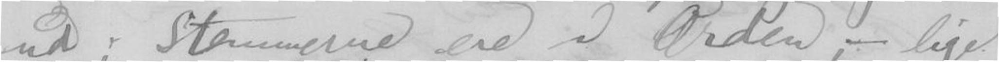ud; Stemmerne ere i Orden; - lige
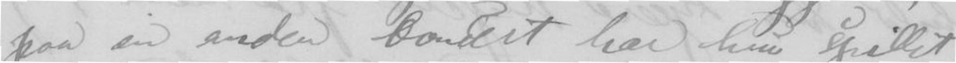paa en anden Consert har hun spillet
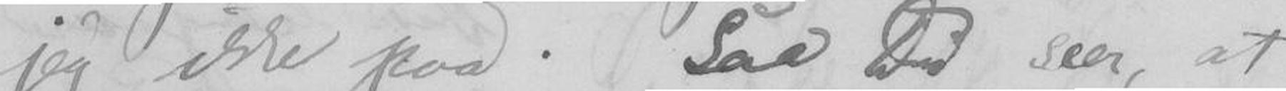jeg ikke paa. Saa Du seer, at
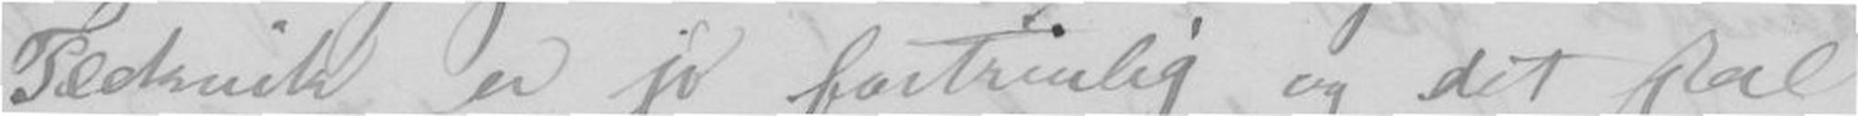Tecknik er jo fortrinlig og det skal
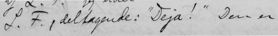L. F., deltagende: "Deja!" Den er
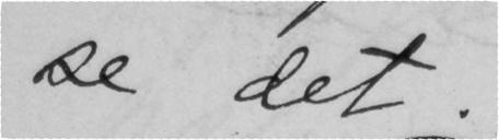se det.
